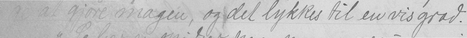ge at gjøre magen, og det lykkes til en vis grad.
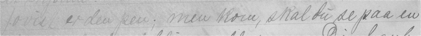"Jovist er den pen; men kom, skal du se paa en
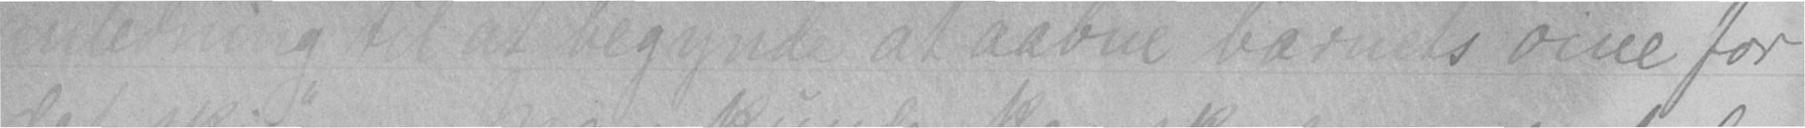anledning til at begynde at aabne barnets øine for
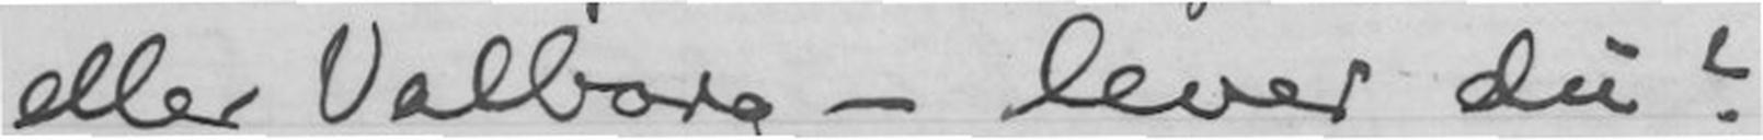eller Valborg - lever du?
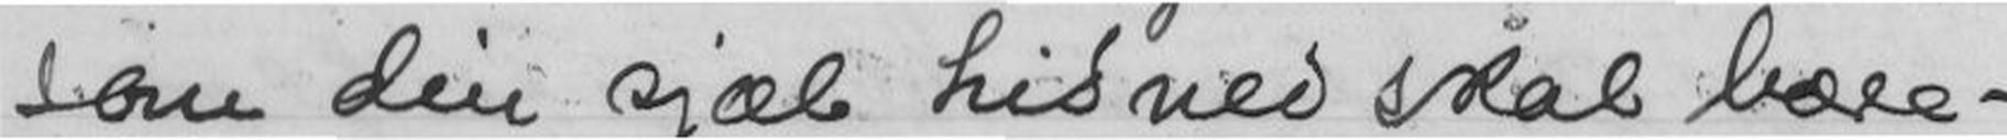som din sjæl hid ned skal bære -
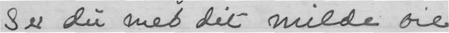Ser du med dit milde øie
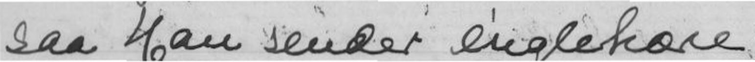saa Han sender engleskare,
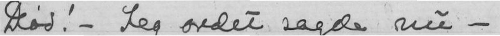Død! - Jeg ordet sagde nu -
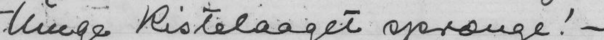- tunge kistelaaget sprænge! -
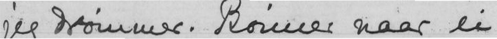jeg drømmer. Bønner naar ei
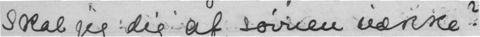Skal jeg dig af søvnen vække?
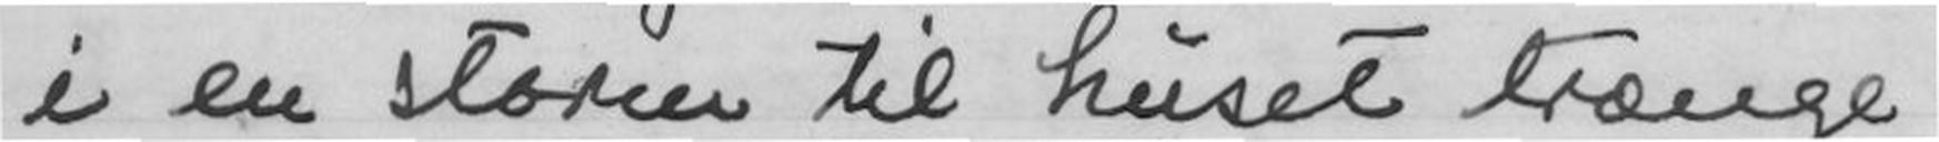i en storm til huset trænge
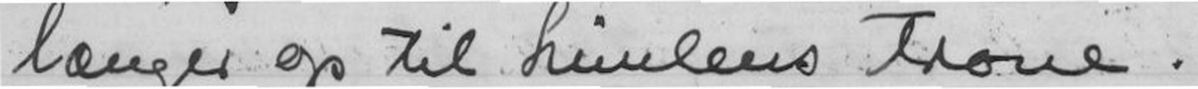længer op til himlens trone.
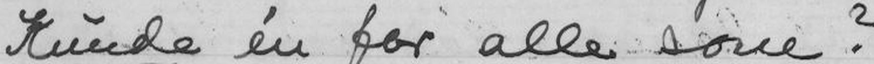Kunde én for alle sone?
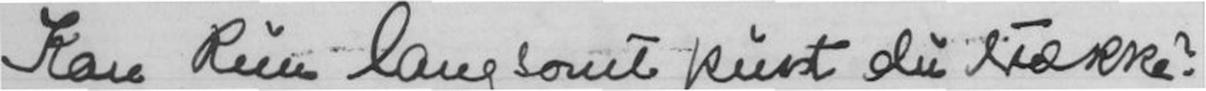Kan kun langsomt pust du trække?
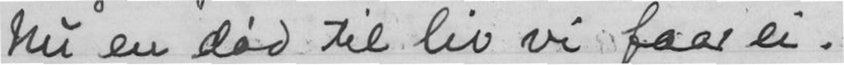Nu en død til liv vi faar ei. -
